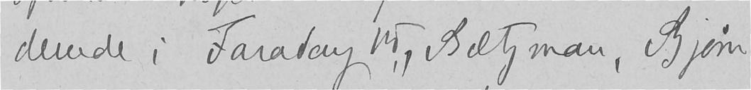derude i Faraday 15, Betzman, Bjørn
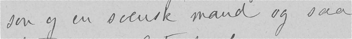son og en svensk mand og saa
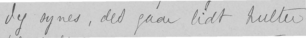Jeg synes, det gaar lidt hulter,
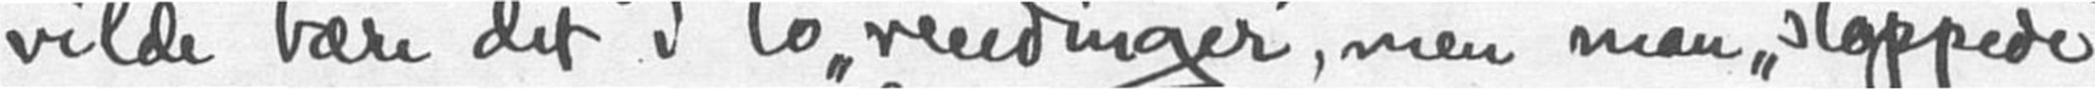vilde bære det i to "vendinger", men man "stoppede"
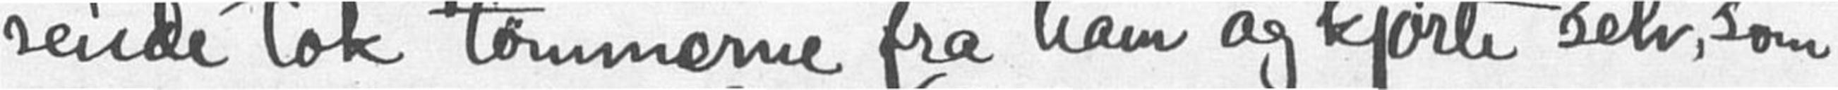sende tok tommerne fra ham og kjørte selv, som
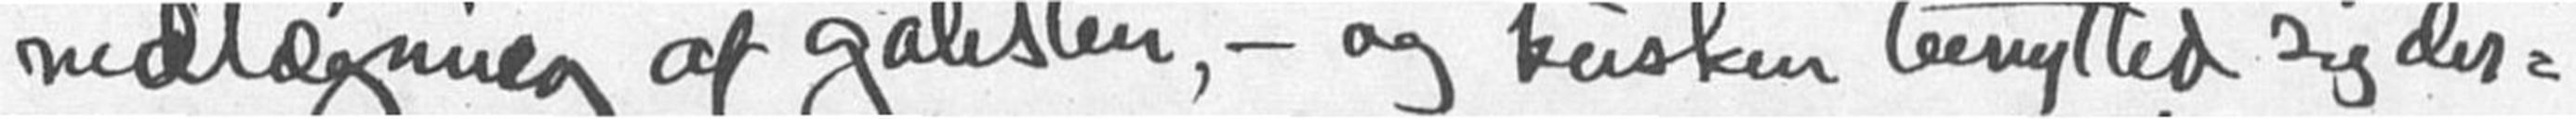nedlægning af gatesten, - og kusken benyttede sig der
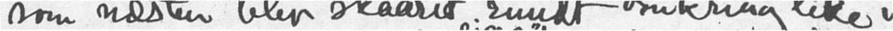som næsten blev skaaret rundt omkring like i
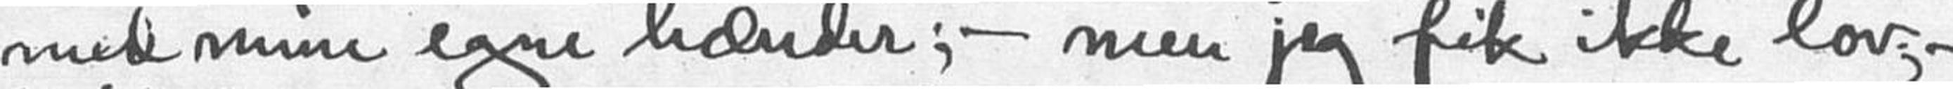med mine egne hænder; - men jeg fik ikke lov:
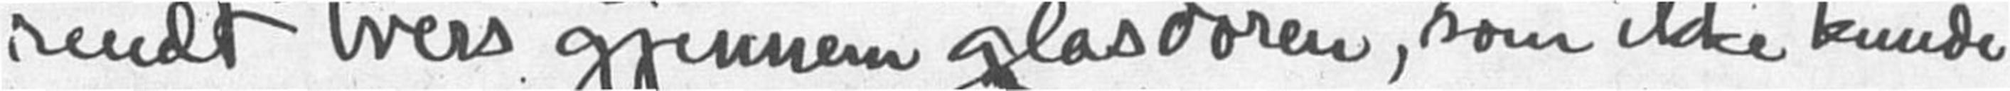rendt tvers gjennem glasdøren, som ikke kunde
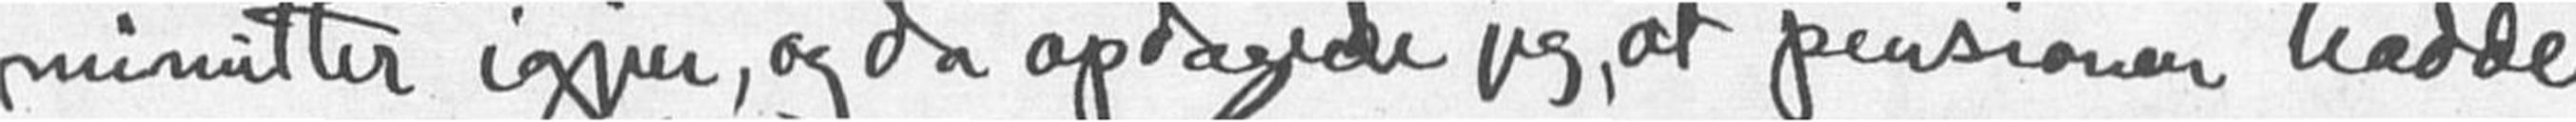minutter igjen, og da opdagede jeg, at pensionen hadde
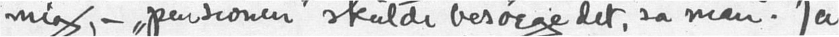mig, - "pensionen" skulde besorge det", sa man. Ja,
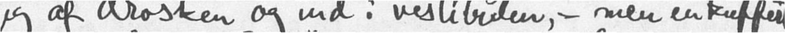jeg af drosken og ind i vestibulen, - men en kuffert
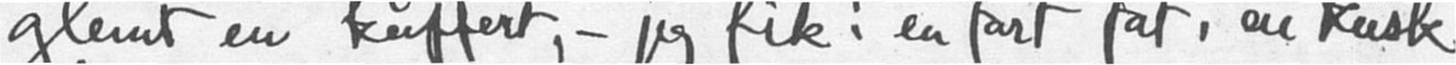glemt en kuffert, - jeg fik i en fart fat i en kusk
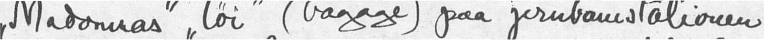"Madonnas" "tøi" (bagage) paa jernbanestationen
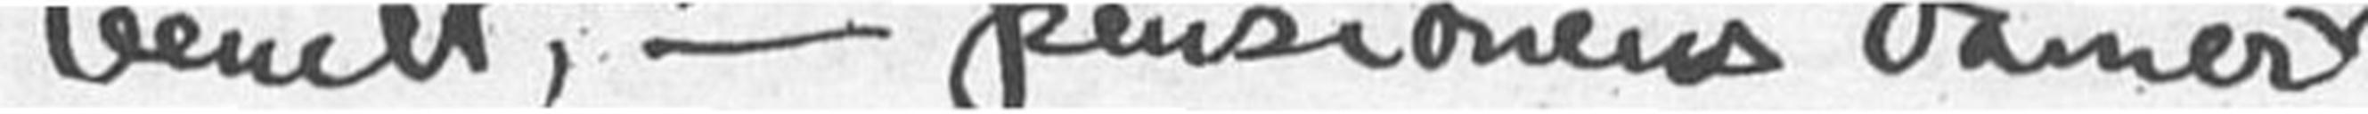benet, - pensionens damer
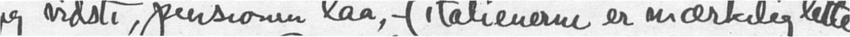jeg vidste, pensionen laa, - (italienerne er mærkelig lette
